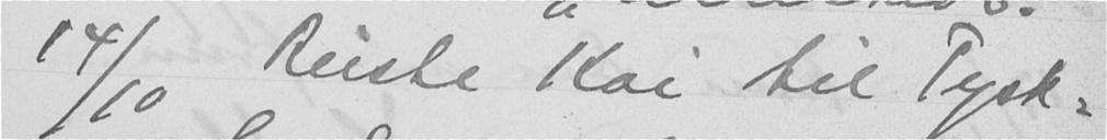14/10 Reiste Kai til Tysk-
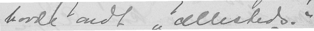havde ondt "allesteds."
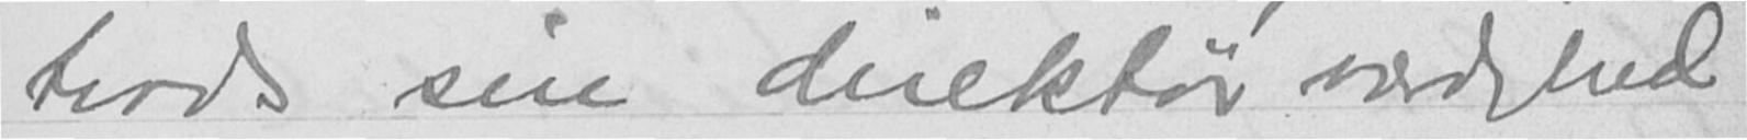trods sin direktør værdighed
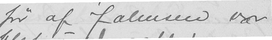før af Johansen var
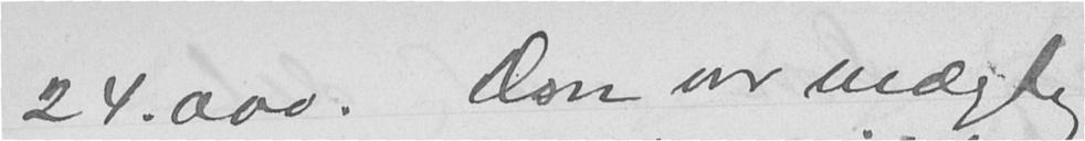24.000. Don var mægtig
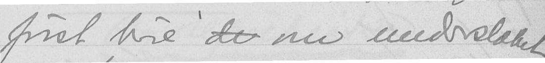først høre de om underslæbet
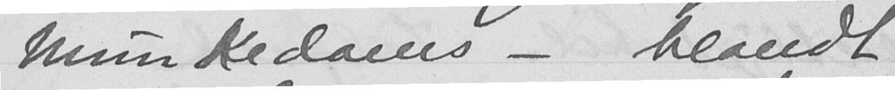Munkedams - blandt
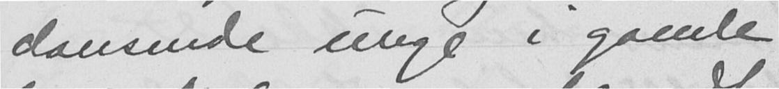dansende unge i gamle
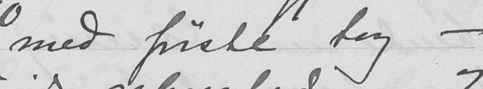med første tog -
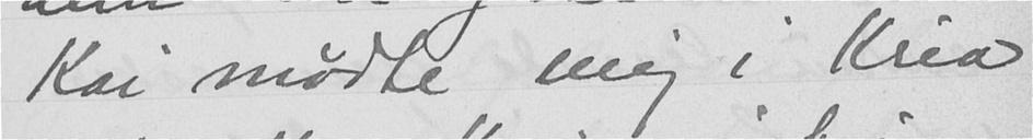Kai mødte mig i Kria
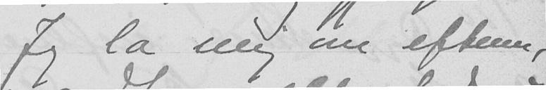Jeg la mig om efterm,
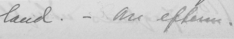land. - Om efterm.
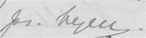pr. tlgm.
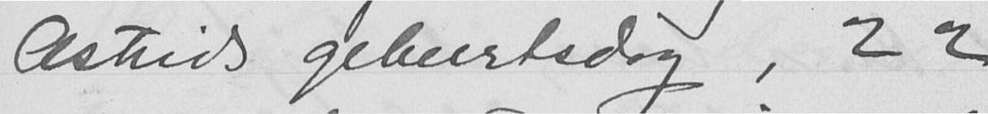Astrids geburtsdag, 22
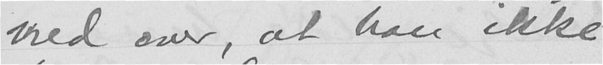vred over, at han ikke
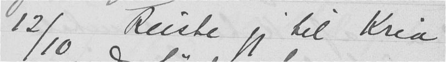12/10 Reiste jeg til Kria
